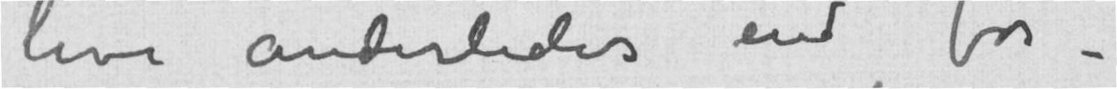leve anderledes end før –
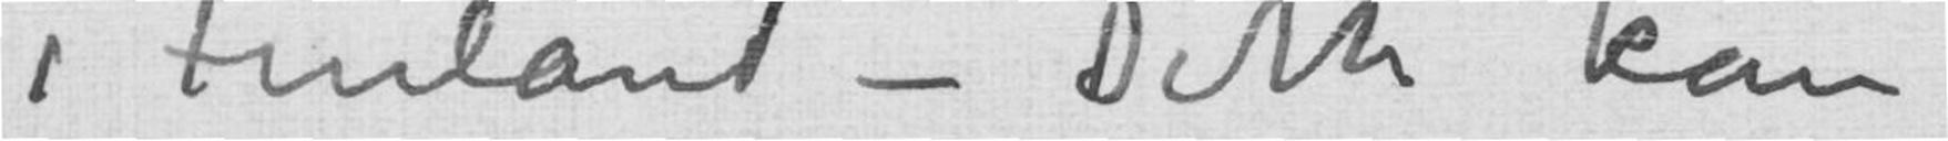i Finland – Dette kan
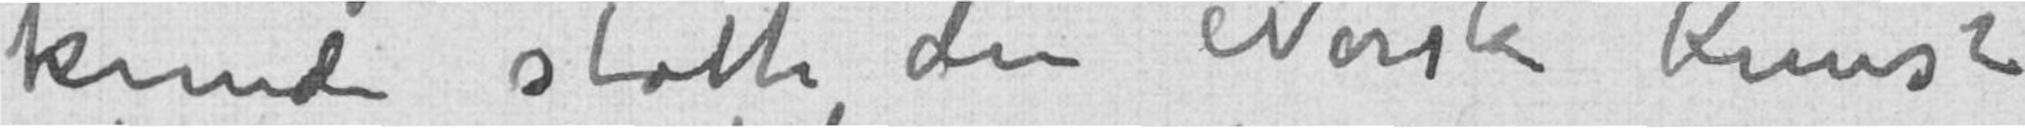kunde støtte den Norske Kunst
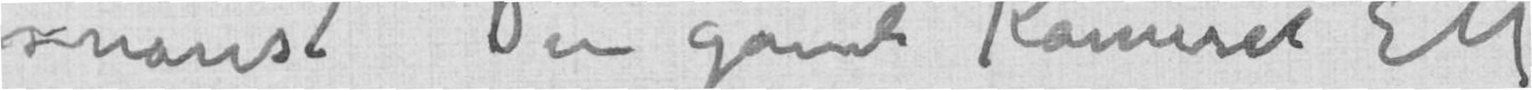snarest Din gamle Kamerat E M
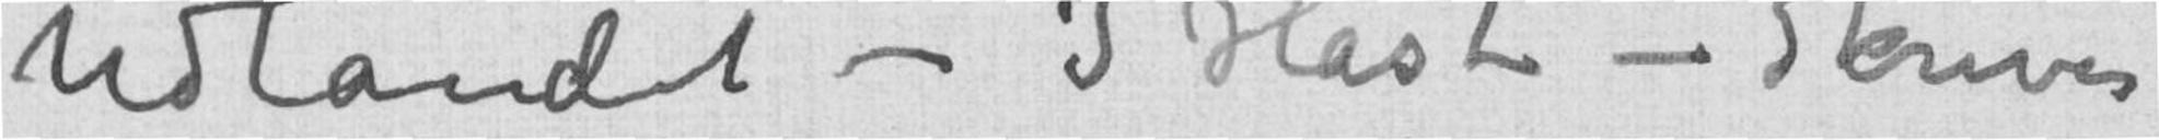Udlandet – I Hast – Skriver
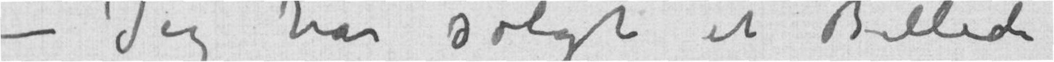– Jeg har solgt et Billede
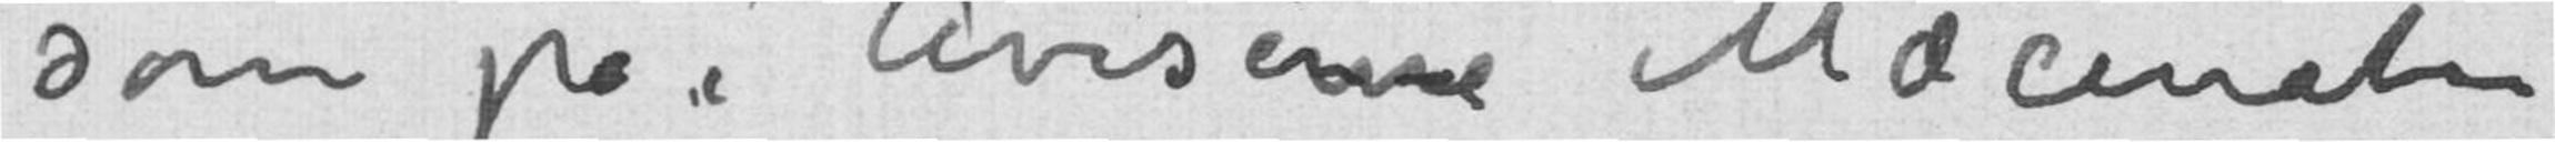som på i aviser – Mæcenaten
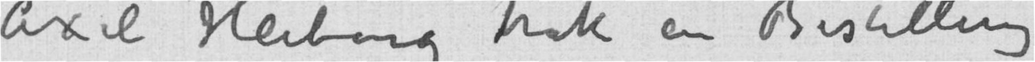Axel Heiberg trak en Bestilling
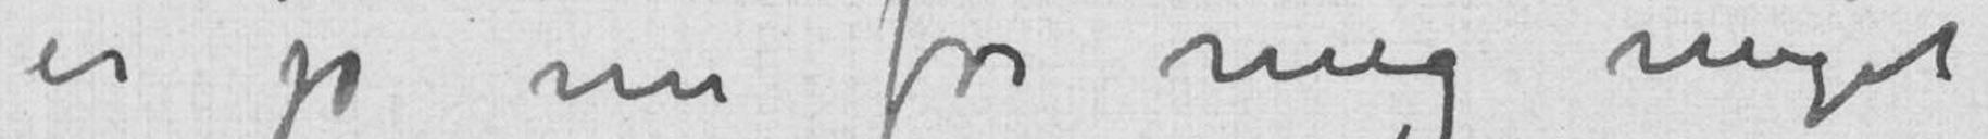er jo nu for mig meget
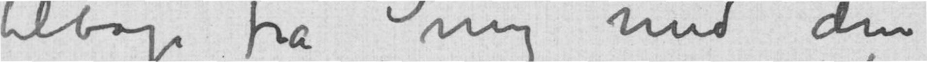tilbage fra mig med den
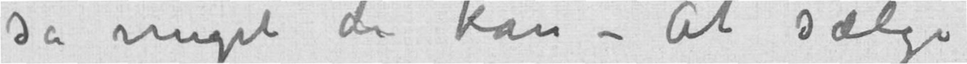så meget de kan – At sælge
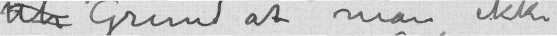Ut Grund at man ikke
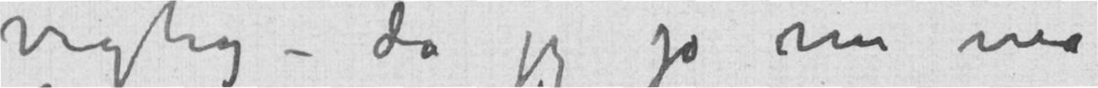vigtig – da jeg jo nu må
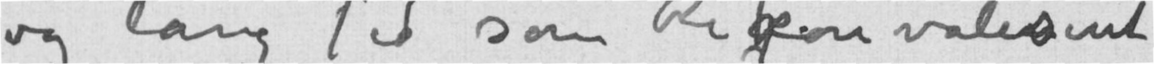og lang Tid som Rekonvalesent
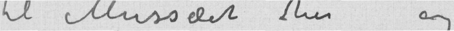til Musæet her og
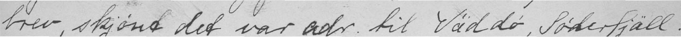brev skjønt det var adr til Väddø, Søderfjäll.
